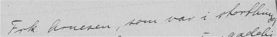Frk. Arnesen, som var i storthinget,
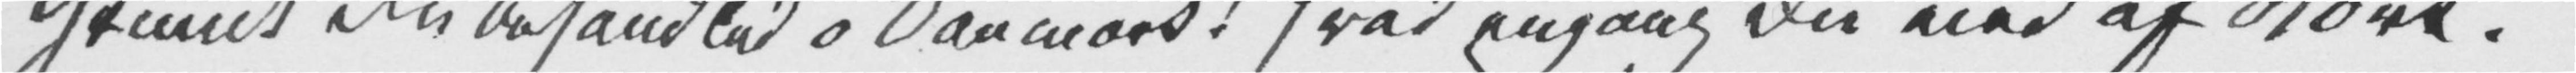Grumt du behandled o Danmark! Hvad engang du eied af Stort.
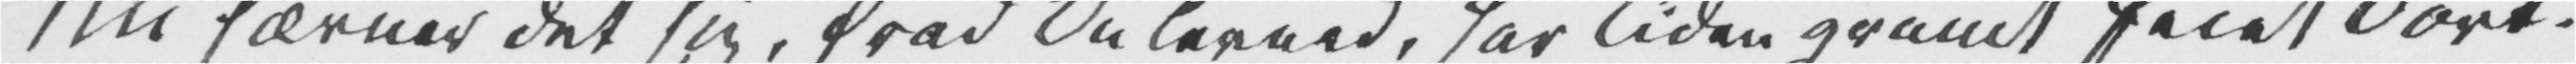Nu hævner det sig, hvad Du levned, har Tiden grumt feiet bort.
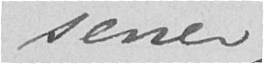sener
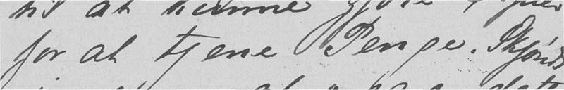for at tjene Penge. Skjøndt
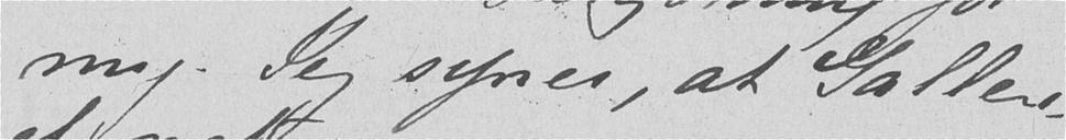mig. Jeg synes, at Galleri-
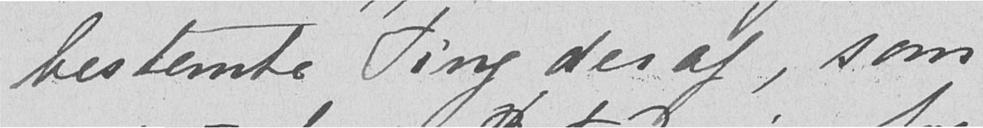bestemte Ting deraf, som
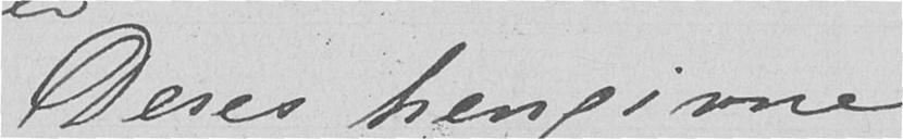Deres hengivne
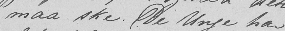maa ske. De Unge har
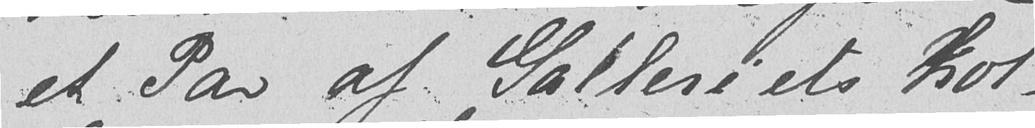et Par af Galleriets Hol-
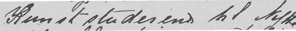Kunst-studerende til Nytte
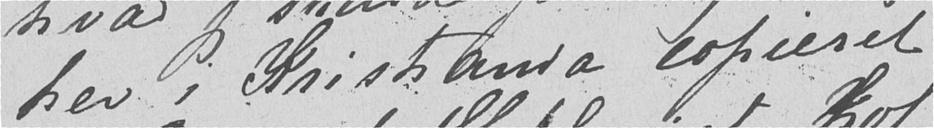her i Kristiania copieret
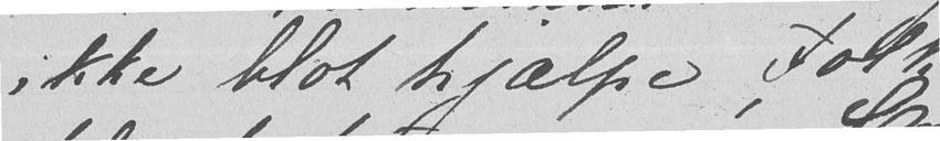ikke blot hjælpe Folk
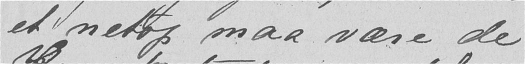et netop maa være de
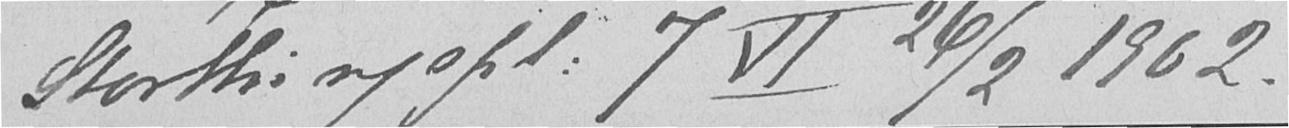Storthingspl. 7 VI 26/2 1902.
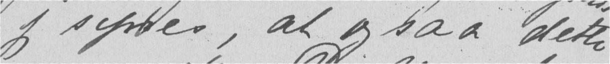jeg synes, at ogsaa dette
